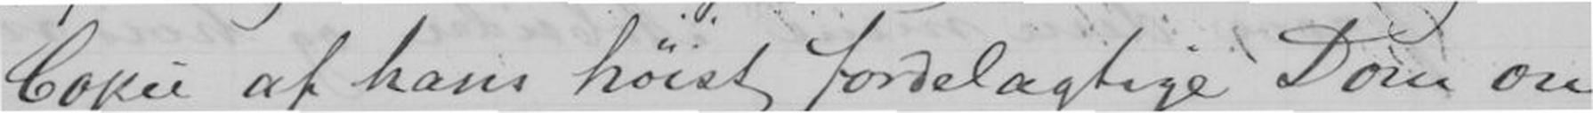Copi af ham høist fordelagtige Dom om
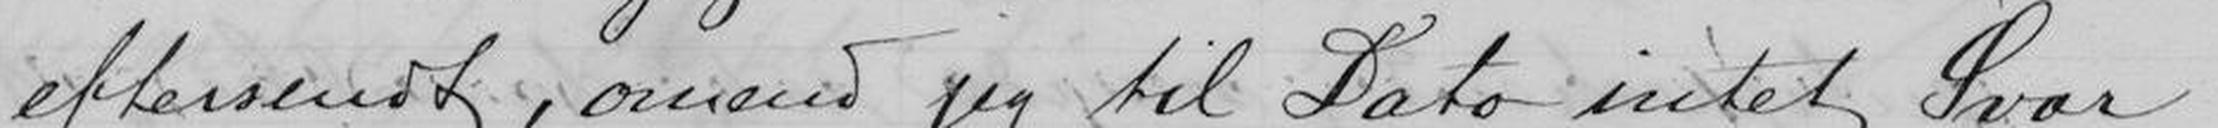eftersendt, omend jeg til Dato intet Svar
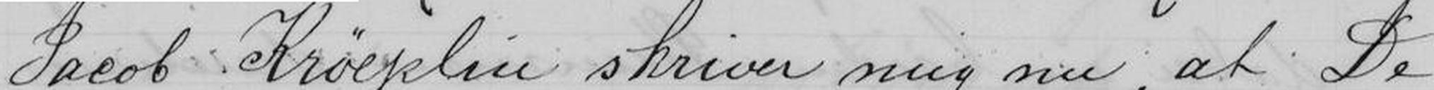Jacob Kröeplin skriver mig nu at De
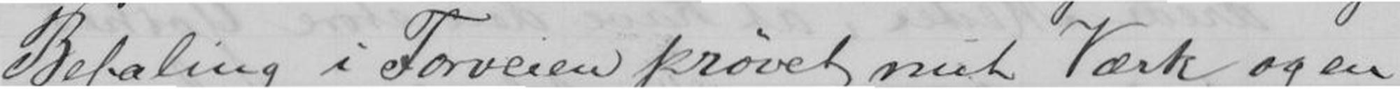Befaling i Forveien prøvet mit Værk og en
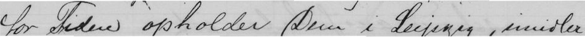for Tiden opholder Dem i Leipzig, imidler-
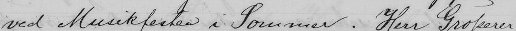ved Musikfesten i Sommer. Herr Großerer
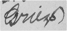Orelis)
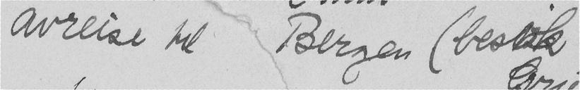avreise til Bergen (besøk
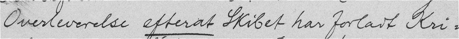Overleverelse efterat Skibet har forladt Kri-
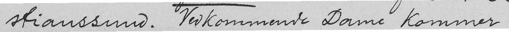stianssund. Vedkommende Dame kommer
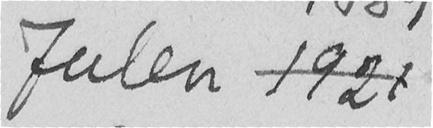Julen 1921
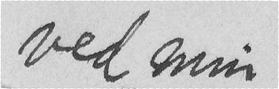ved min
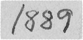1889
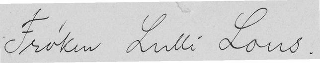Frøken Lulli Lous.
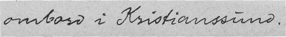ombord i Kristianssund.
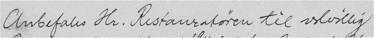Anbefales Hr. Restauratøren til velvillig
